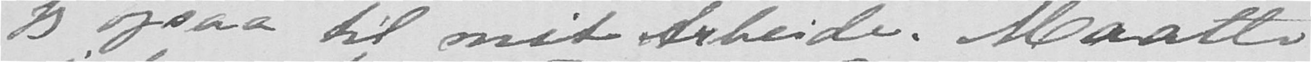jeg ogsaa til mit Arbeide. Maatte
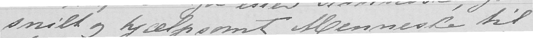snilt og hjuelpsomt Menneske til
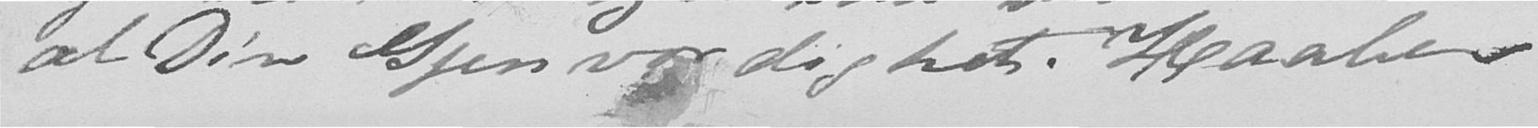at Din Gjenvordighet. Haaber
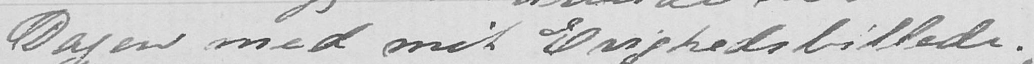Dagen med mit Evighedsbillede.
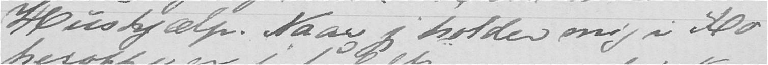Hushjælp. Naar jeg holder mig i Ro
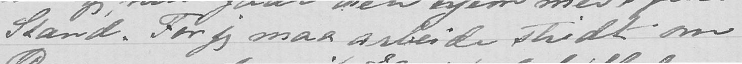Stand. For jeg maa arbeide stridt om
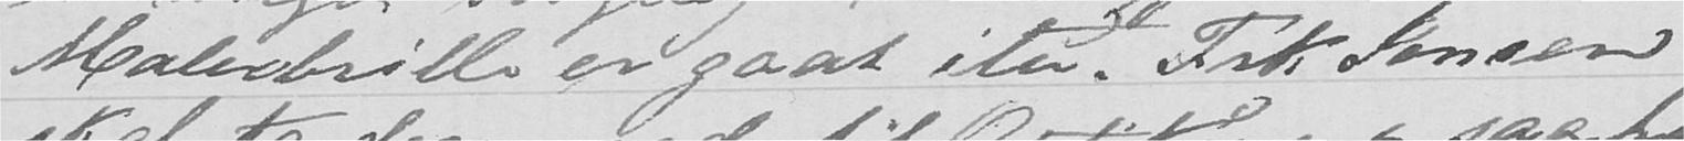Malerbrille er gaat itu. Frk Jonsen
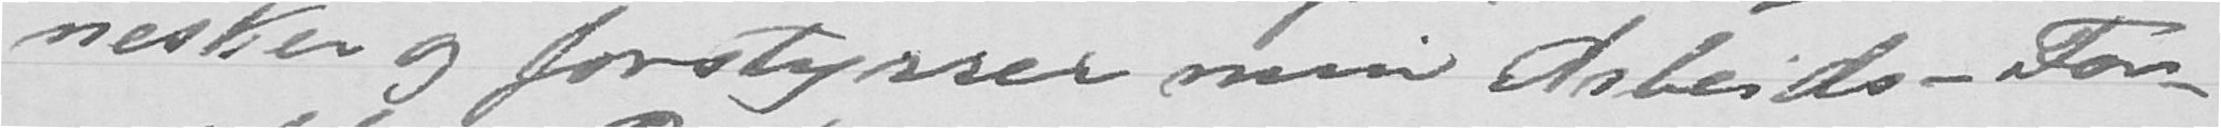nesker og forstyrrer min Arbeids-For-
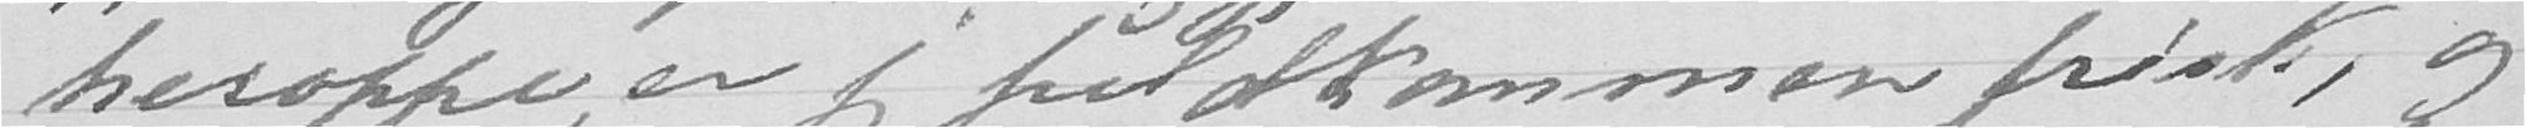heroppe, er jeg fuldkommen frisk, og
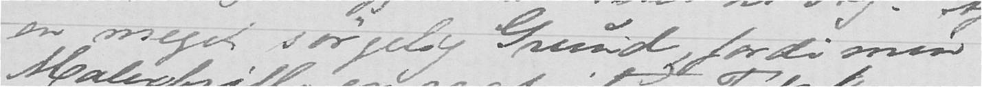en meget sørgelig Grund, fordi min
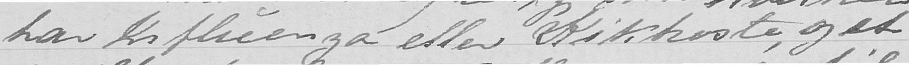har Influenza eller Kikhoste, og et
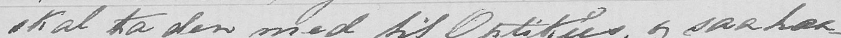skal ta den med til Optikus, og saa haa-
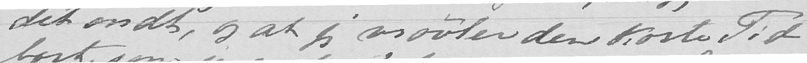det ondt, og at jeg vrøvler den korte Tid
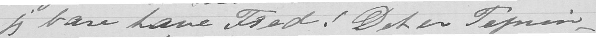jeg bare have Fred! Det er Tegnin-
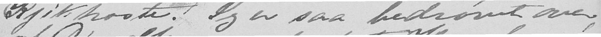Kjikhoste! Jeg er saa bedrøvet over,
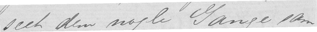seet dem nogle Gange sam-
